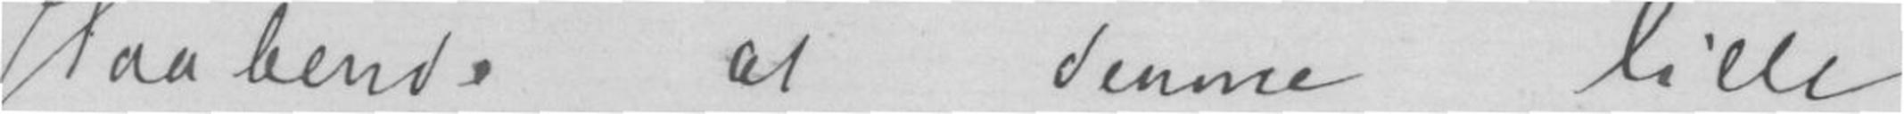Haabende at denne lille
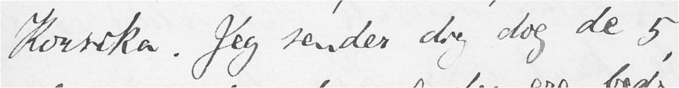Korsika. Jeg sender dig dog de 5,
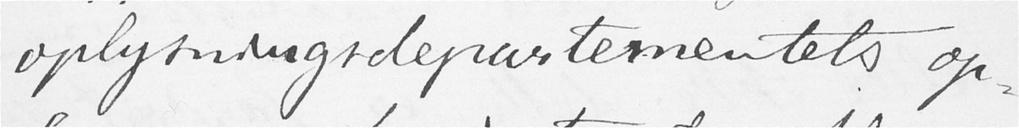oplysningsdepartementets op-
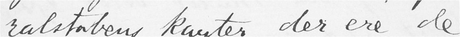ralstabens karter, der ere de
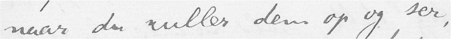naar du ruller dem op og ser,
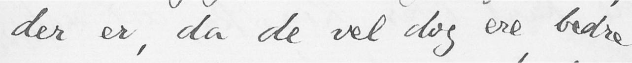der er, da de vel dog ere bedre
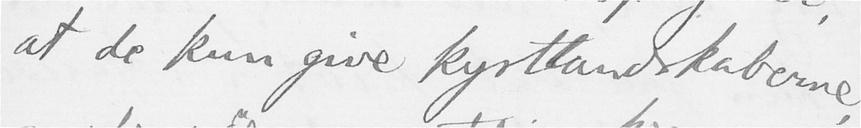at de kun give kystlandskaberne,
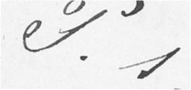P. s.
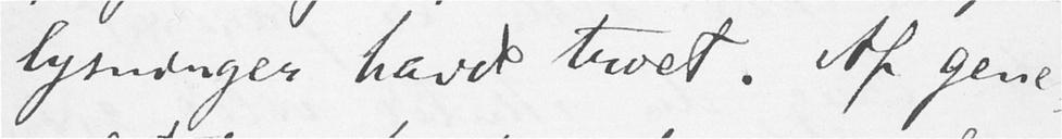lysninger havde troet. Af gene-
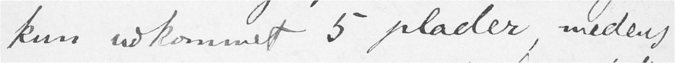kun udkommet 5 plader, medens
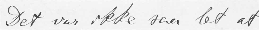Det var ikke saa let at
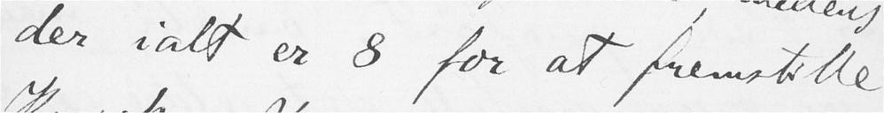der ialt er 8 for at fremstille
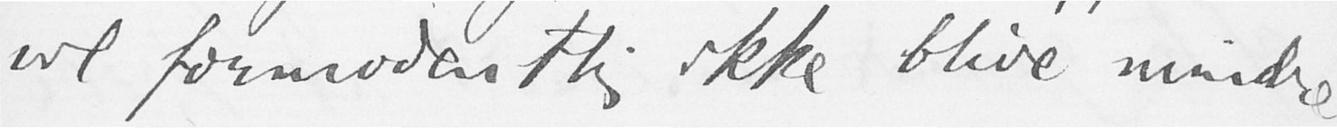vil formodentlig ikke blive mindre,
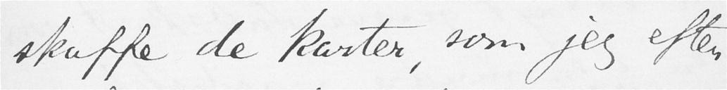skaffe de karter, som jeg efter
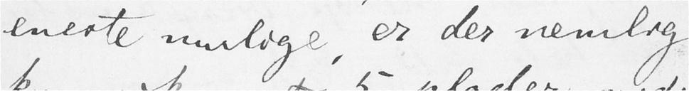eneste mulige, er der nemlig
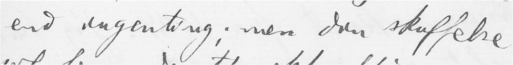end ingenting; men din skuffelse
